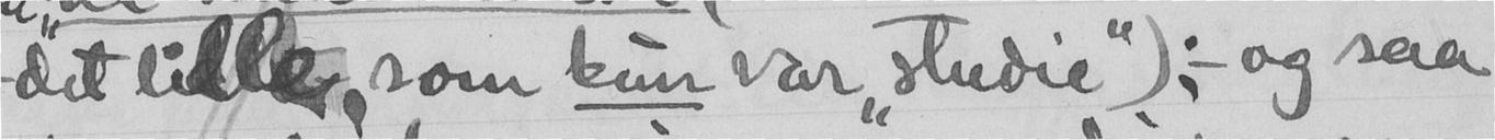det lille, som kun var "studie"); - og saa
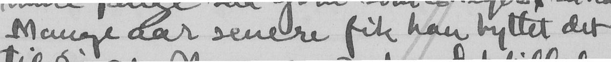Mange dar senere fik han byttet det
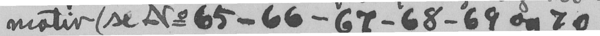motiv (se No 65 - 66 - 67 - 68 - 69 og 70
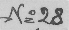No 28
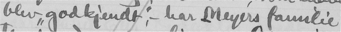blev "godkjendt", - har Meyers familie
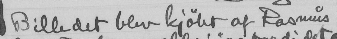Bille det blev kjøbt af Rasmus
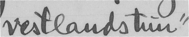vestlandstun"
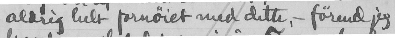aldrig helt fornøiet med dette, - førend jeg
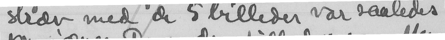stræv med de 5 billeder var saaledes
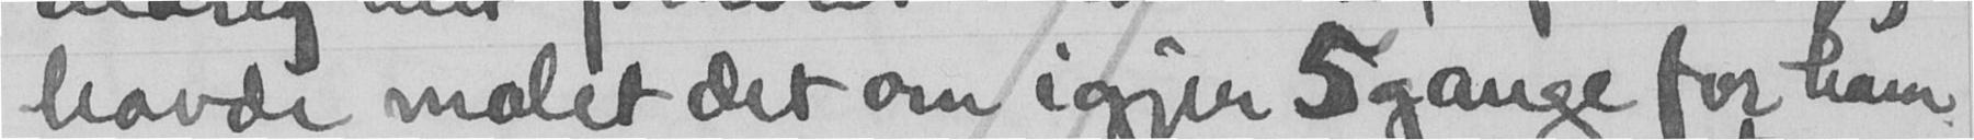havde malet det om igjen 5 gange for ham
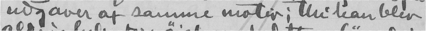udgaver af samme motiv; thi han blev
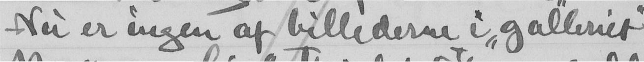Nu er ingen af billederne i "galleriet"
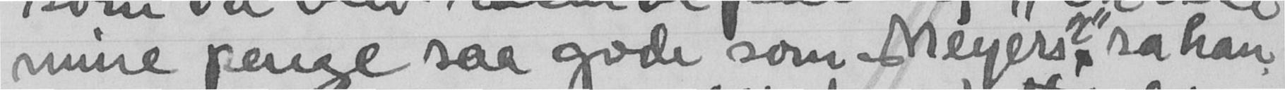mine penge saa gode som Meyers?" sa han
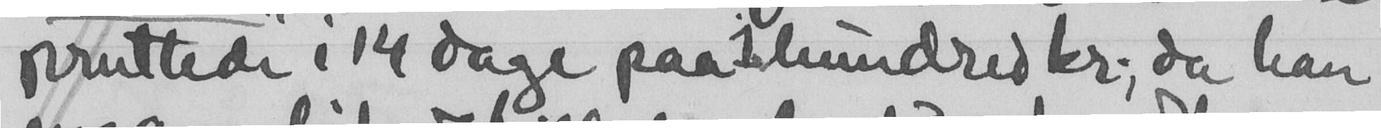pruttede i 14 dage paa hundred kr., da han
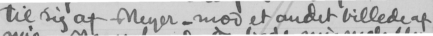til sig af Meyer - mod et andet billede af
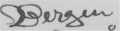Bergen
